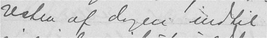resten af dagen indtil
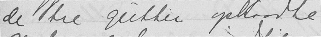de tre gutter ophaadte
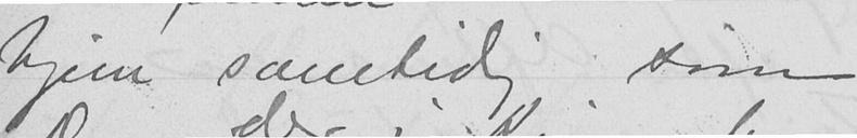hjem samtidig som
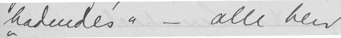"badendes" - alle blev
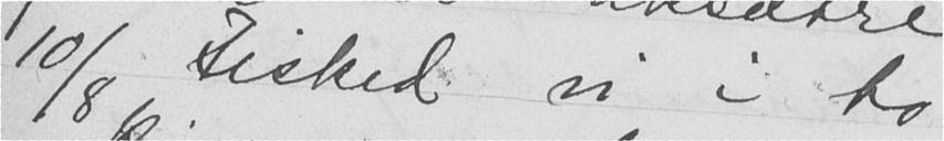10/8 Fisked vi i to
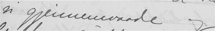vi gjennemvaade og
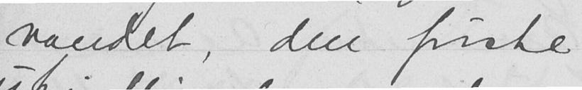vandet, den første
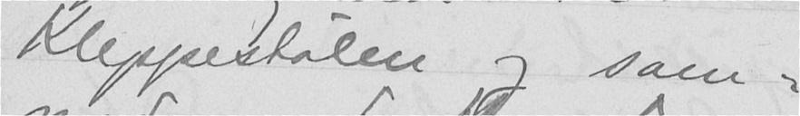Kleppestølen og sam-
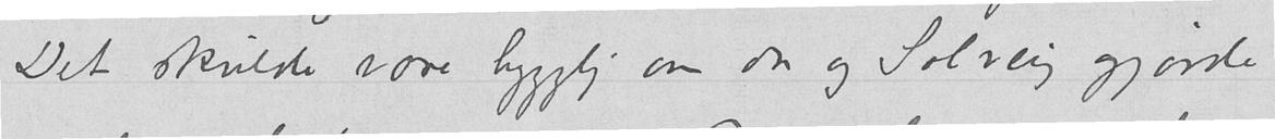Det skulde være hygglig om du og Solveig gjorde
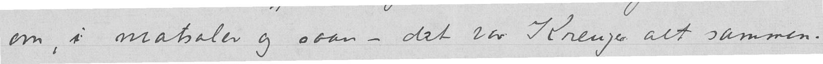om, i matsaler og saan - det var Kreuger alt sammen.
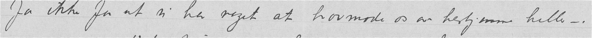Ja ikke for at vi har noget at hovmode os av herhjemme heller – .
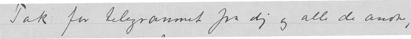Tak for telegrammet fra dig og alle de andre,
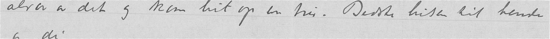alvor av det og kom hit op en tur.  Bedste hilsen til hende
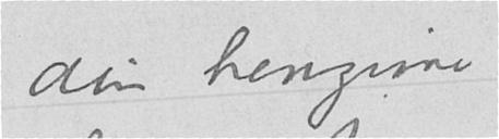din hengivne
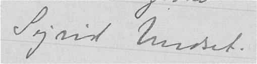Sigrid Undset.
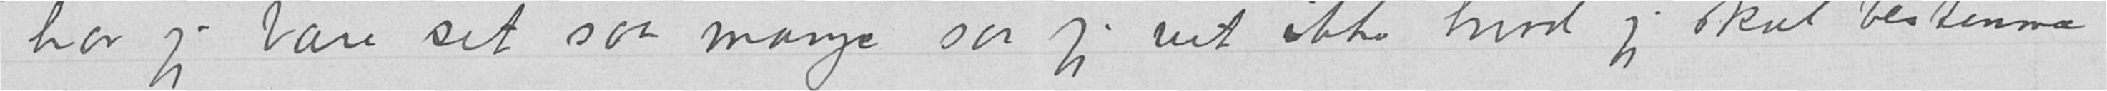har jeg bare set saa mange saa jeg vet ikke hvad jeg skal bestemme
